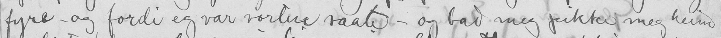fyre - og fordi eg var vortne vaate - og bad meg pikte meg heim
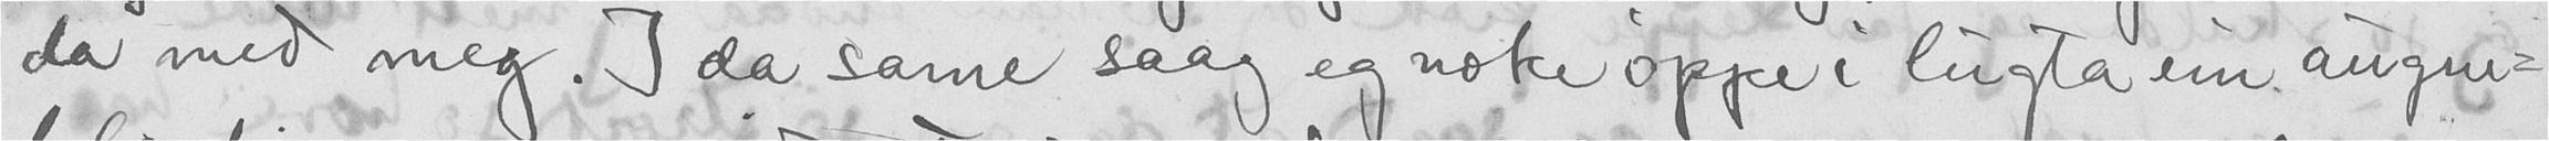da med mig. I da same saag eg noke oppe i lugta um augne-
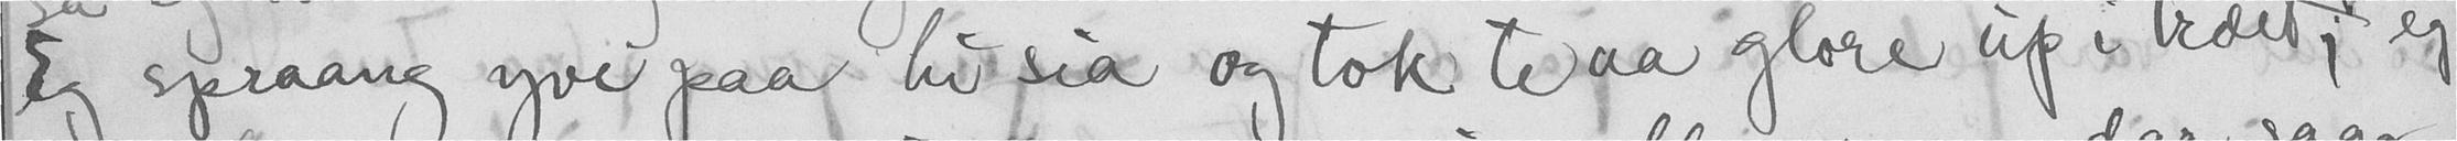Eg spraang yve paa hi sia og tok te aa glore up i træet, eg
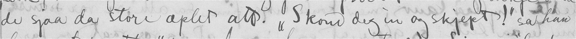de sjaa da store æplet att. "Skom deg in og skjept!" sa han
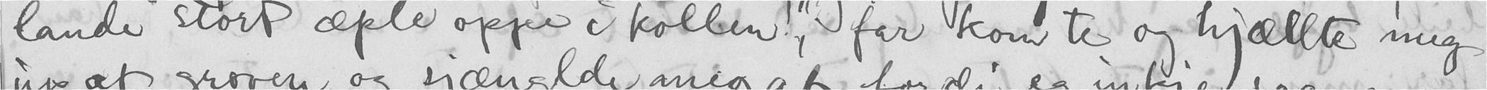landi stort æple oppe i kollen!", far kom te og hjællte mig
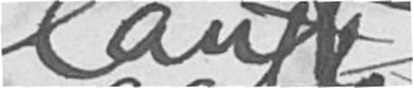lautt
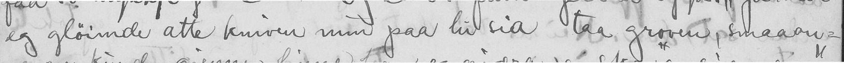eg gløimde atte kniven min paa lu sia taa groven, smaa an-
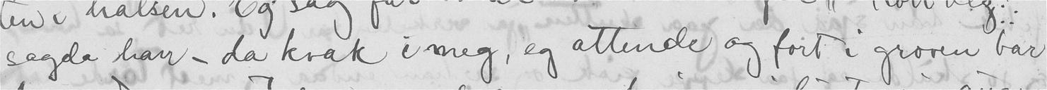sagde han - da kvak i mig, og attende og fort i groven bar
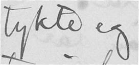tykte eg
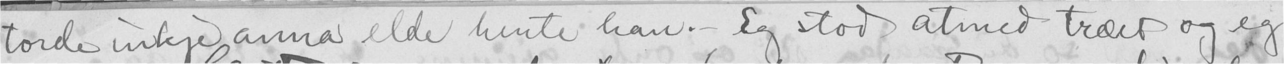torde inkje, anna elde hente han. Eg stod atmed træet og eg
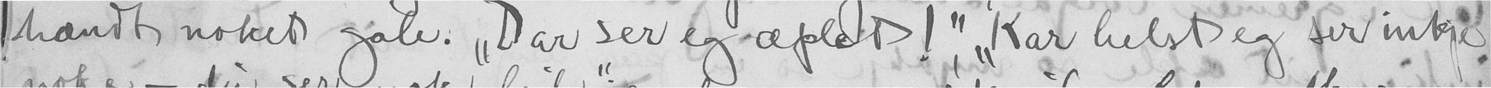hændt noket gale: "Dar ser eg æplet!", "Kor helst eg ser inkje
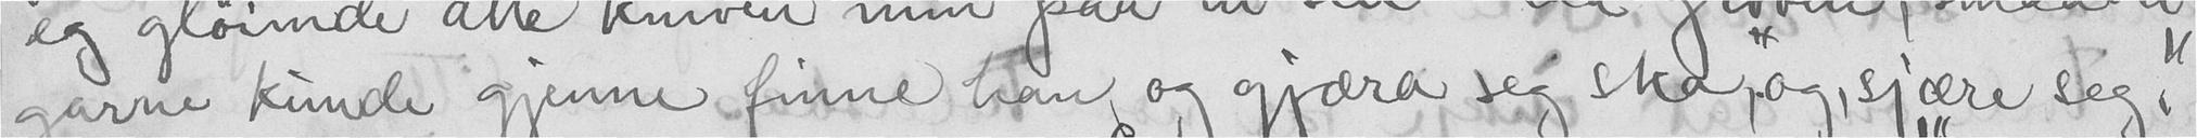garne kunde gjenne finne han og gjæra seg ska, og, sjære sig!
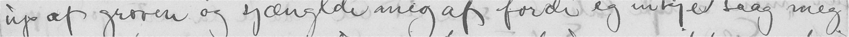up af groven og sjænglde mig af fordi eg inkje saa mig
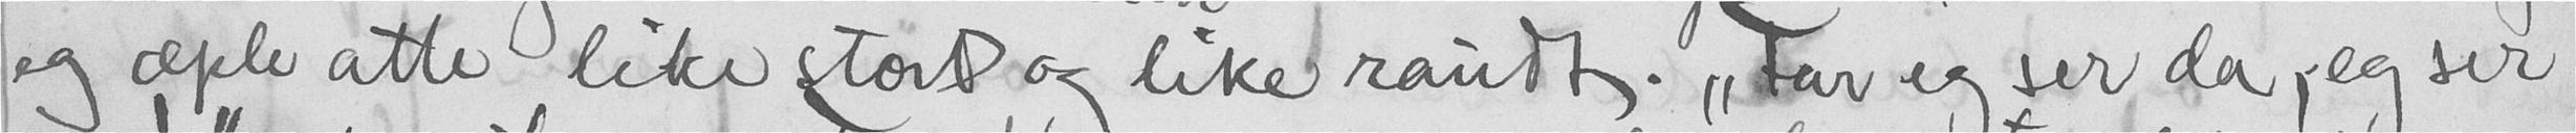eg æple atte like stort og like raudt. "Far eg ser da, eg ser
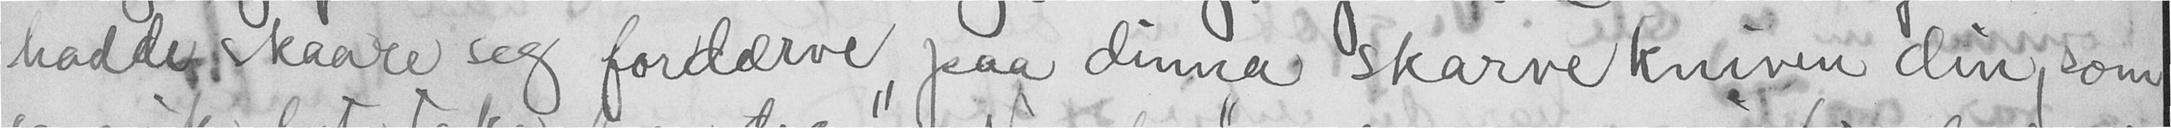hadde skaare seg fordærve paa dinna skarve kniven din, som
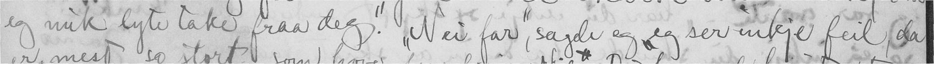eg nuk lyte take fraa deg." "Nei far", sagde eg "eg ser inkje feil, da
